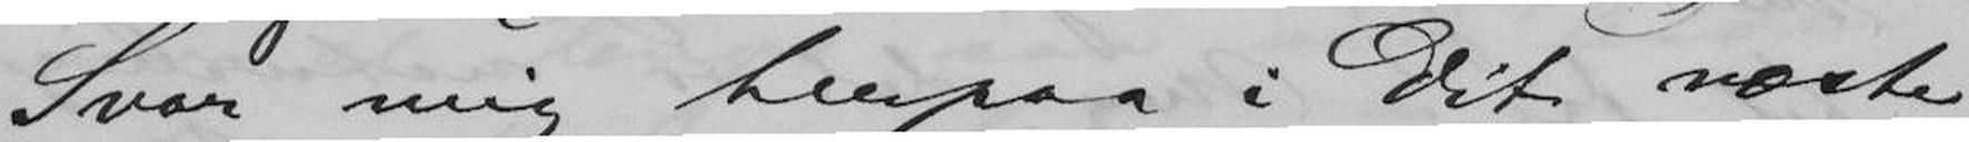Svar mig herpaa i Dit næste
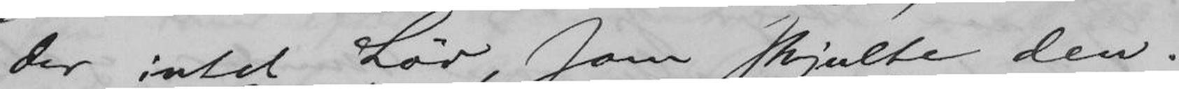der intet Løv, som skjulte den.
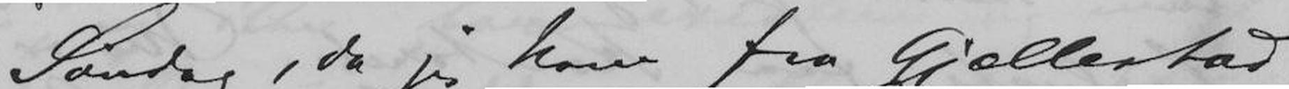Søndag, da jeg kom fra Gjællestad
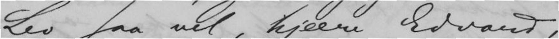Lev saa vel, kjære Edvard,
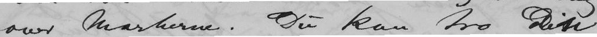over Markerne. Du kan tro Din
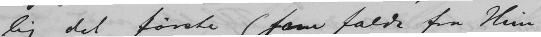lig det første (som faldt fra Him-
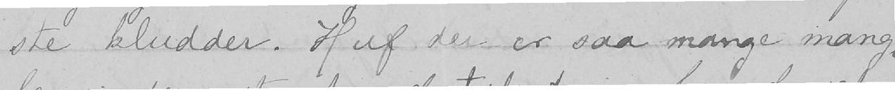ste kludder. Huf der er saa mange mang-
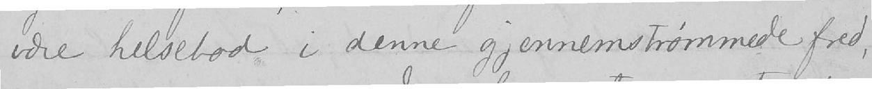være helsebod i denne gjennemstrømmede fred,
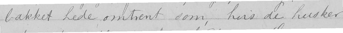bakket hede omtrent som, hvis de husker
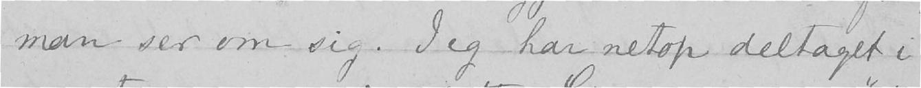man ser om sig. Jeg har netop deltaget i
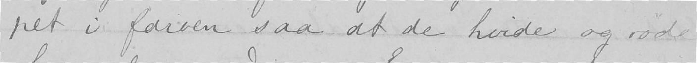pet i farven saa at de hvide og røde
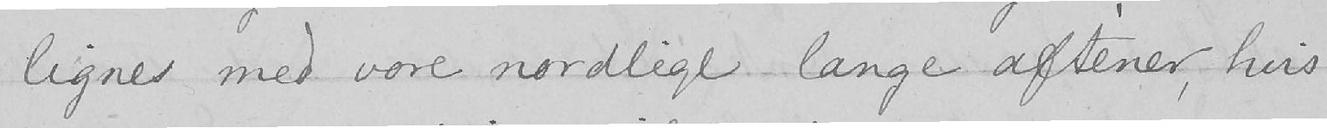lignes med vore nordlige lange aftener, hvis
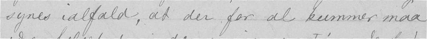synes ialfald, at der for al kummer maa
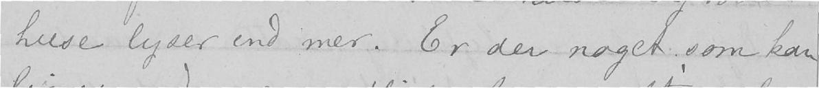huse lyser end mer. Er der noget som kan
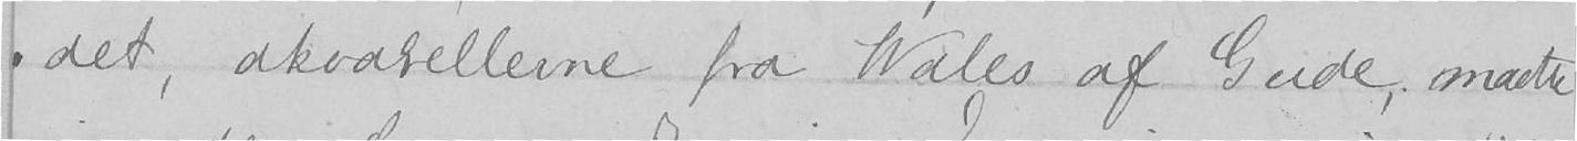det, akvarellerne fra Wales af Gude, maatte
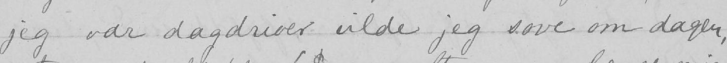jeg var dagdriver vilde jeg sove om dagen,
